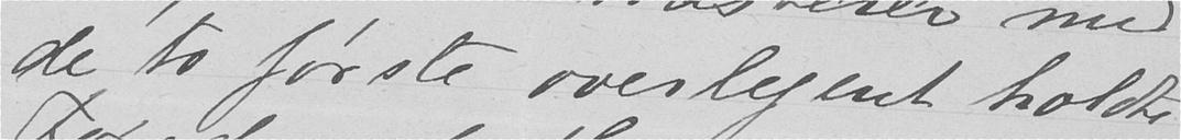de to første overlegent holdte
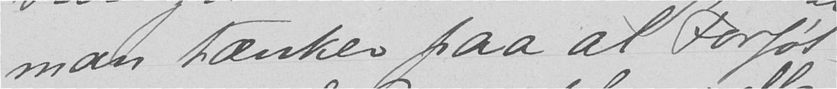man tænker paa al Forføl-
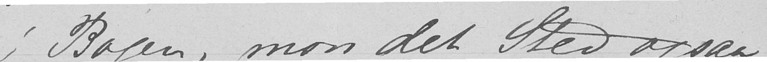i Bogen, mon det Sted ogsaa
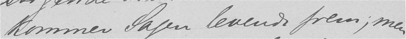kommer Sagen levende frem; men
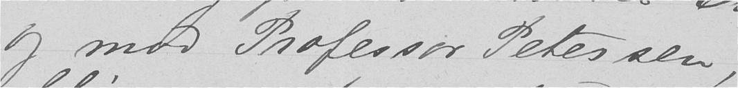og mod Professor Petersen,
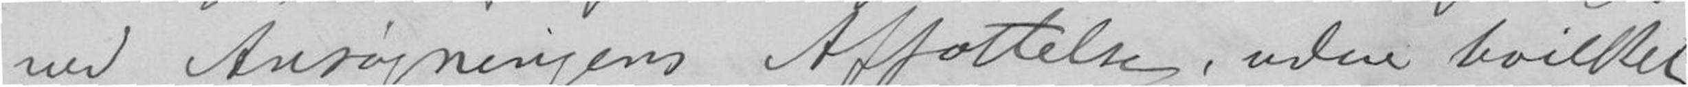ved Ansøgningens Affattelse, uden hvilket
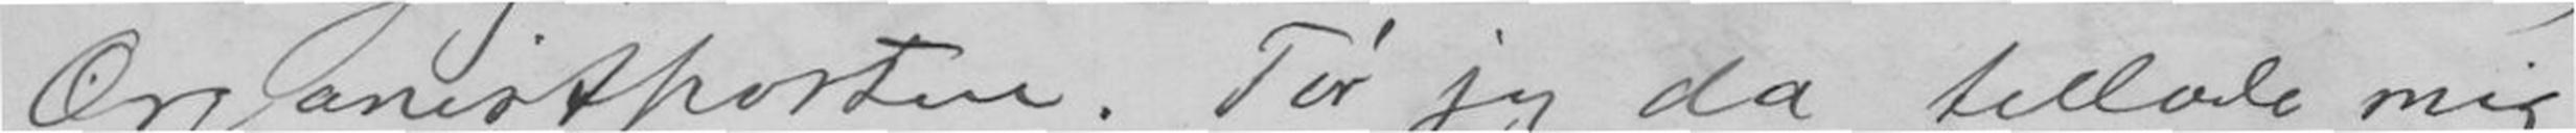Organistposten. Tør jeg da tillade mig
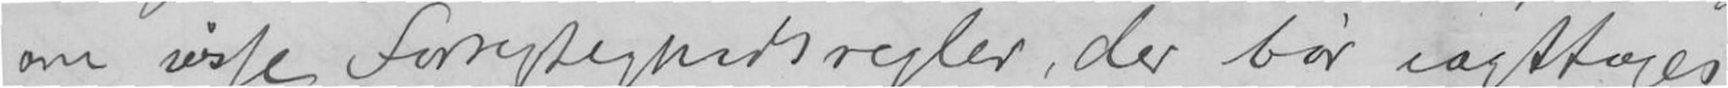om visse Fosigtighedsregler, der bør iagttages
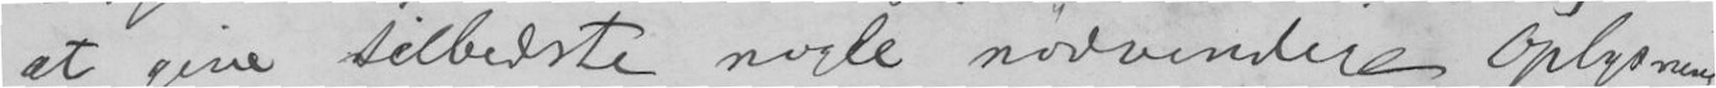at give tilbedste nogle nødvendige Oplysninger
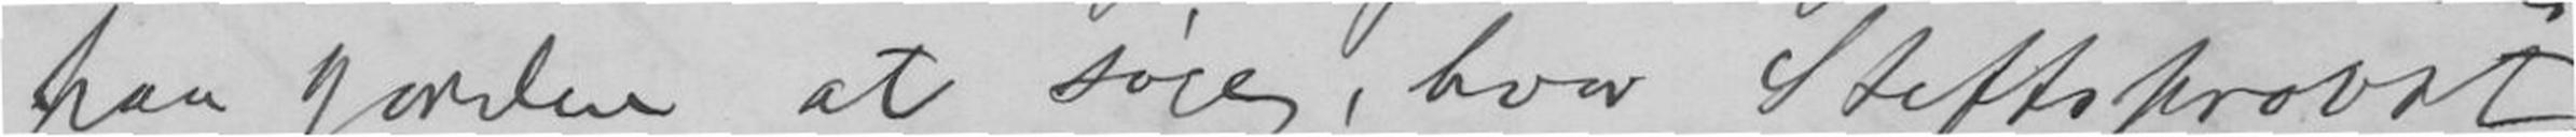paa jorden at søge, hvor Stiftsprovat
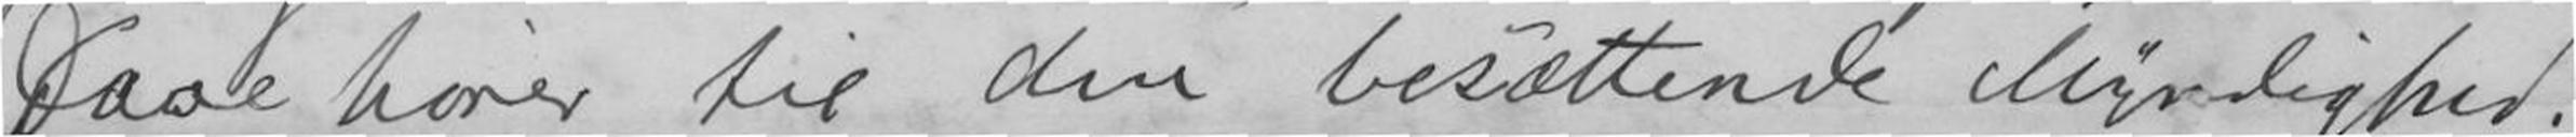Daae hører til den besættende Myndighed.
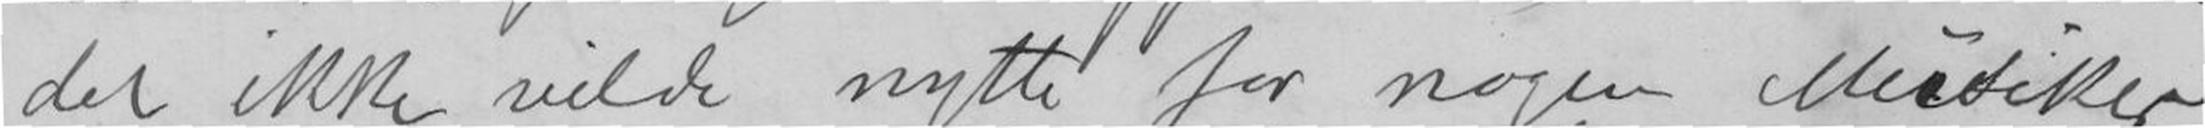der ikke vilde nytte for nogen Musiker
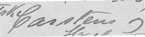Carstens og
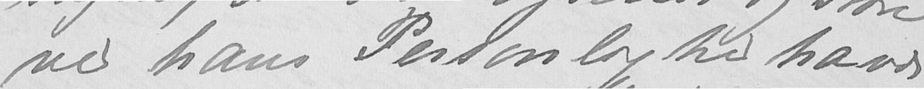ved hans Personlighed havde
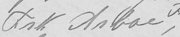Frk Arboe,
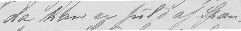da han er fuld af Spæn-
